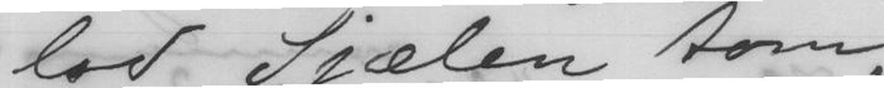lod Skjælen tom.
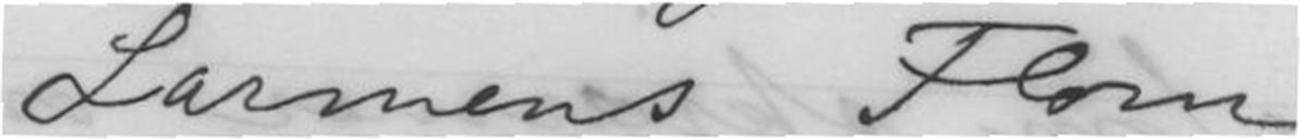Larmens Flom
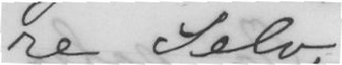re Selv,
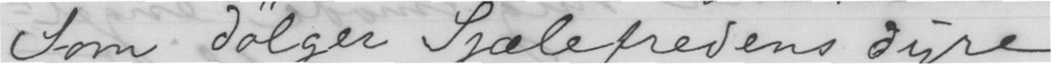Som dølger Sjælefredens dyre
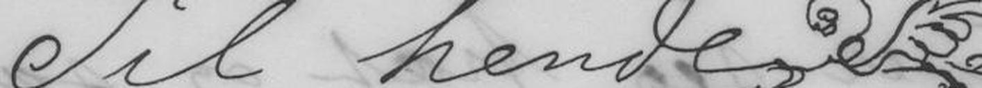Til hende
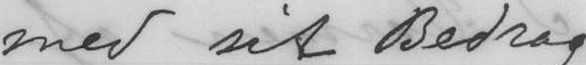med sit Bedrag.
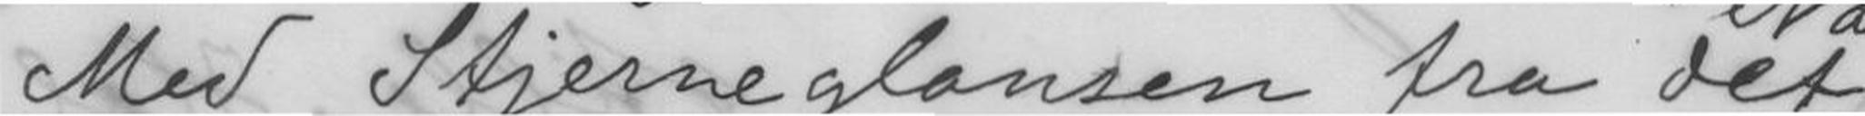Med Stjerneglandsen fra det dun
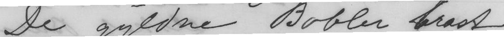De gyldne Bobler brast
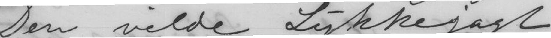Den vilde Lykkejagt
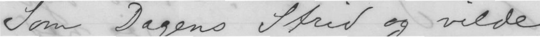Som Dagens Strid og vilde
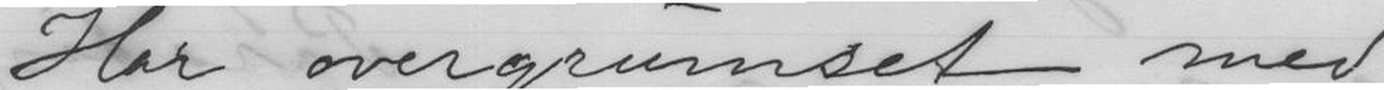Har overgrumset med
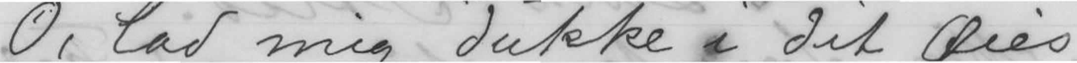O, lad mig dukke i dit Øies
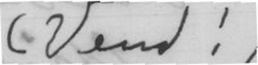(Vend!)
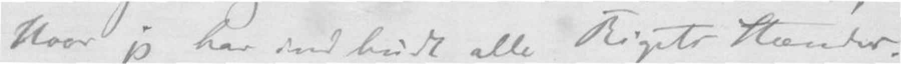Hvor jeg har indbudt alle Rigets Stænder.
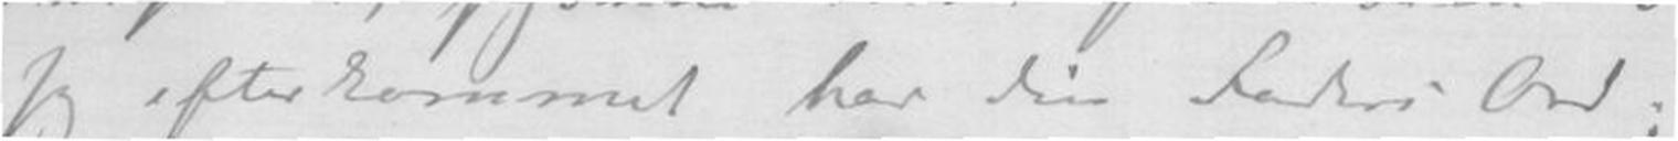Jeg efterkommet har din Faders Ord;
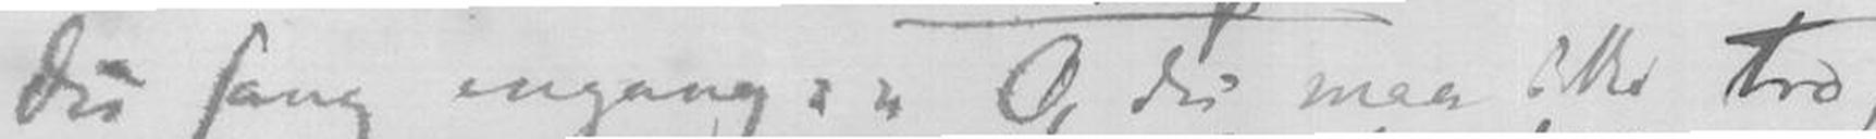Du sang engang: "O, du maa ikke tro,
